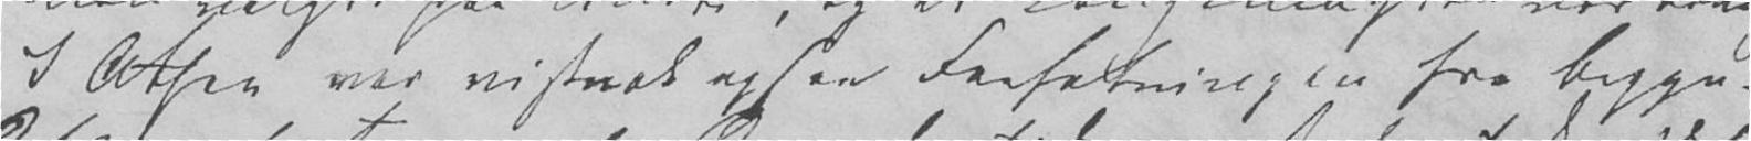I Athen var vistnok ogsaa Forfatningen fra Begyn-
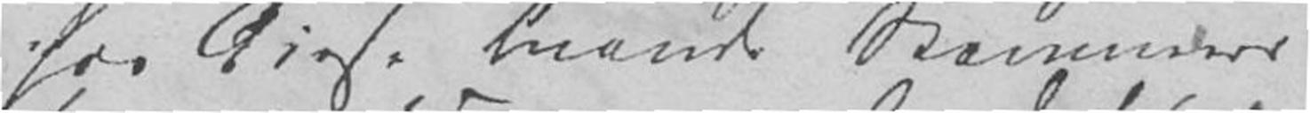hos disse tvende Stammers
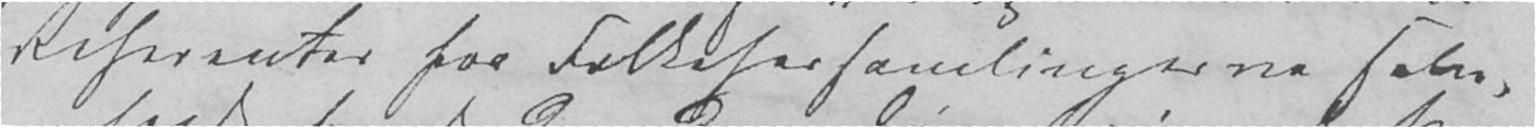Referenter for Folkeforsamlingerne selv,
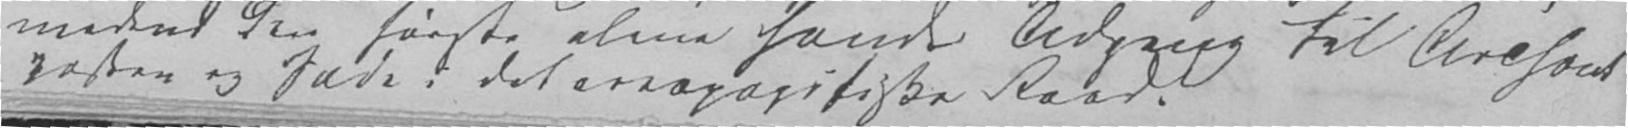medens den første alene havde Adgang til Archont
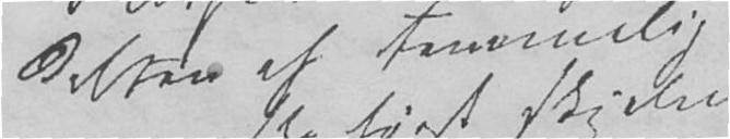delsen af temmelig
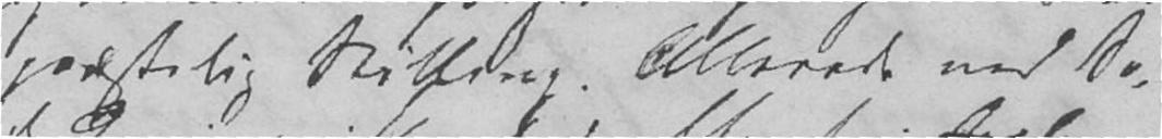præstelig Stilling. Allerede ved So-
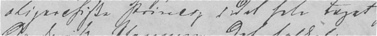oligarchiske Princip i det hele taget
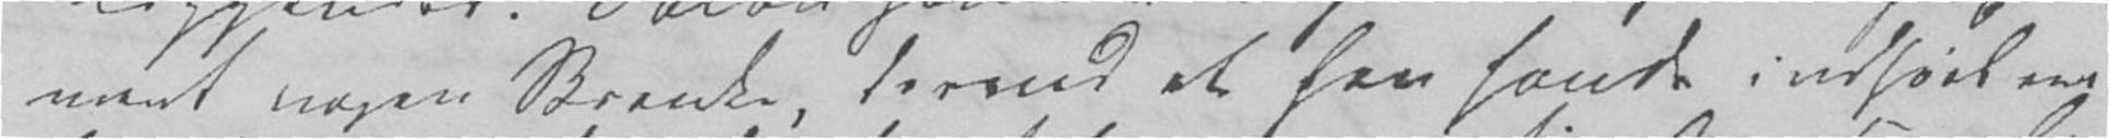ment nogen Skranke, derved at han havde indført en
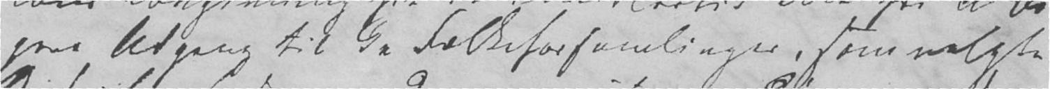gere Adgang til de Folkeforfamlinger, som valgte
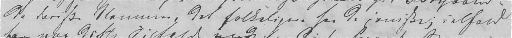de doriske Stammer, det folkeligere hos de ioniske; ialfald
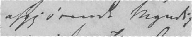afgjørende Myndig-
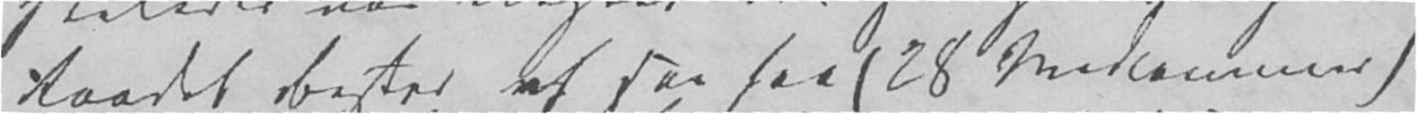Raadet bestod af saa faa (28 Medlemmer),
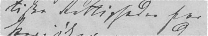tiske Rettigheder for
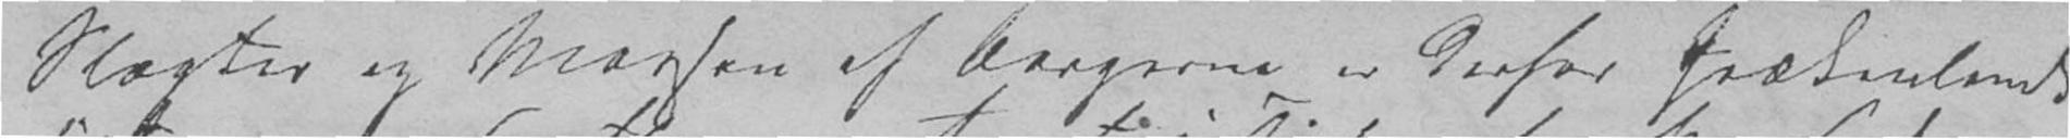Slægter og Massen af Borgerne er derfor Grækenlands
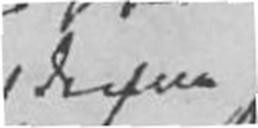denne
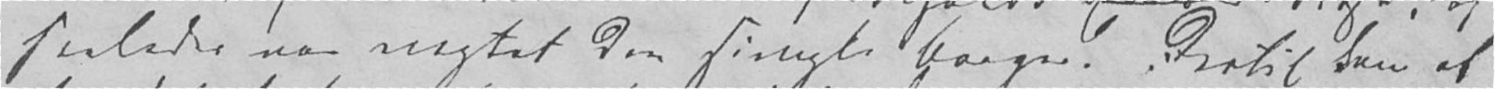saaledes var nægtet den simple Borger. Dertil kom at
